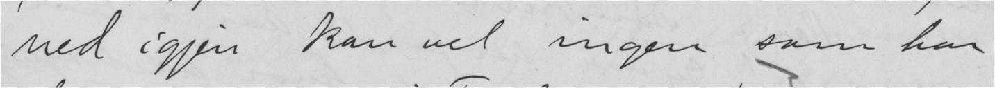ned igjen kan vel ingen som har
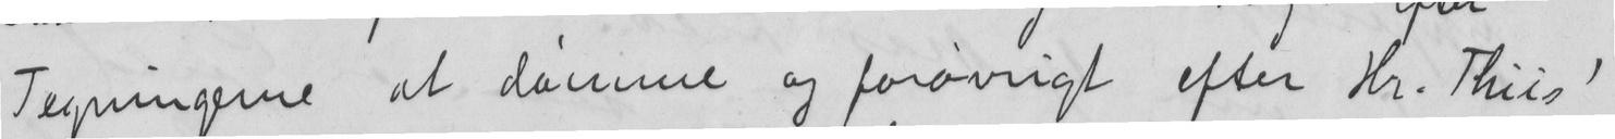Tegningerne at dømme og forøvrigt efter Hr. Thiis'
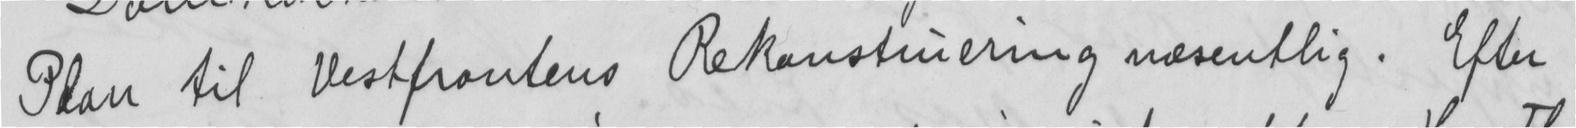Plan til Vestfrontens Rekonstruering væsentlig. Efter
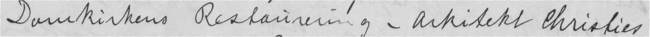Domkirkens Restaurering — Arkitekt Christies
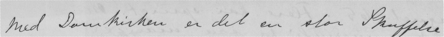Med Domkirken er det en stor Skuffelse
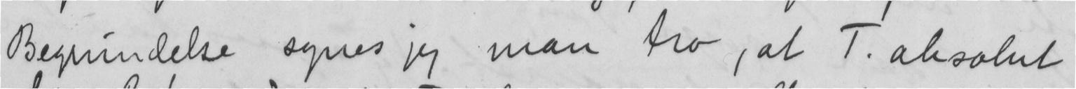Begrundelse synes jeg man tro, at T. absolut
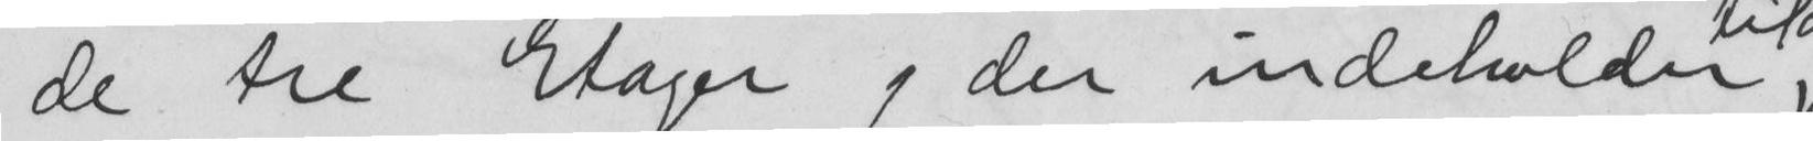de tre Etager, der indeholder
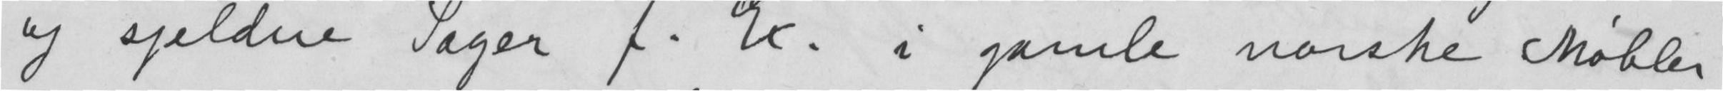og sjeldne Sager f. Ex. i gamle norske Møbler
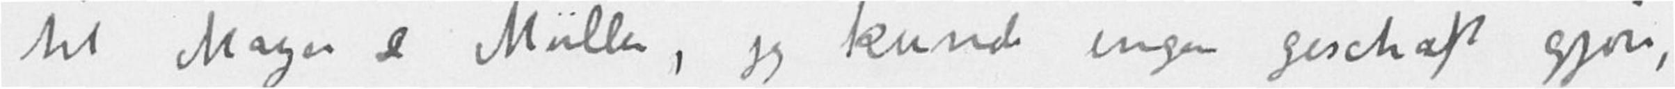til Mayer & Müller, jeg kunde ingen gescheft gjøre,
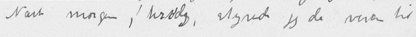Næste morgen, tirsdag, styrede jeg da veien til
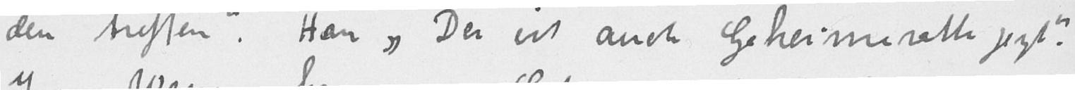den treffen." Han "Der ist auch Geheimeratte jezt."
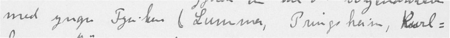med yngre Fysikere (Lummer, Pringsheim, Karl-
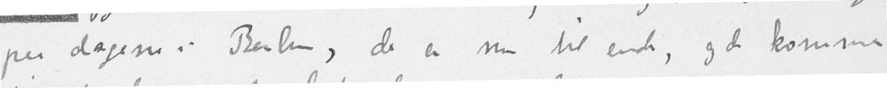paa dagene i Berlin, de er nu til ende, og de kommer
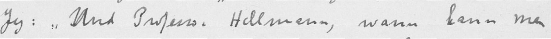Jeg: "Und Professor Hillmann, wann kann man
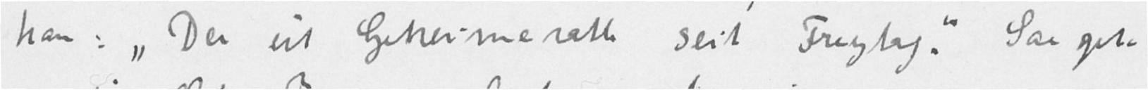han: "Der ist Geheimeratte seit Freytag." Saa gik
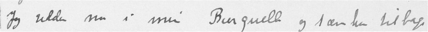Jeg sidder nu i min Burguelle og tænker tilbage
